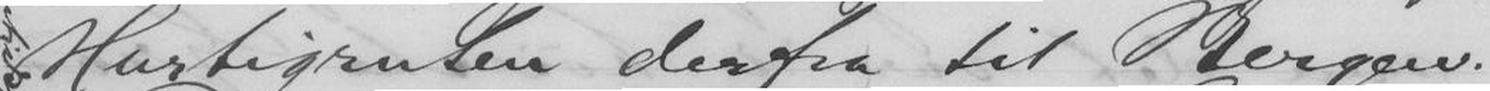Hurtigruten derfra til Bergen.
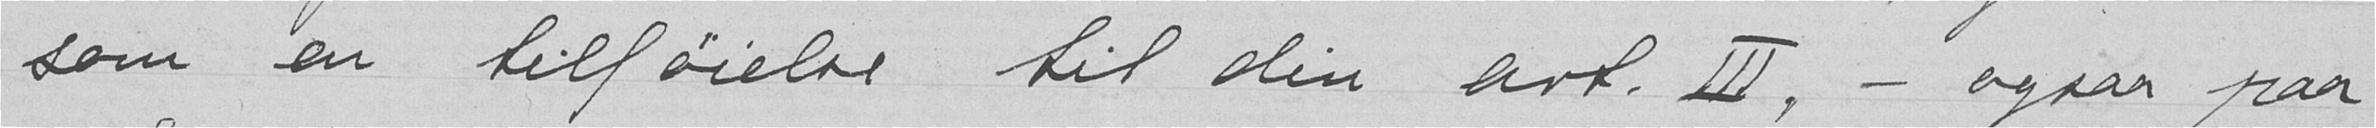som en tilføielse til din art. III, - ogsaa paa
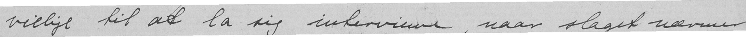villige til at la sig interviewe, naar slaget nærmer
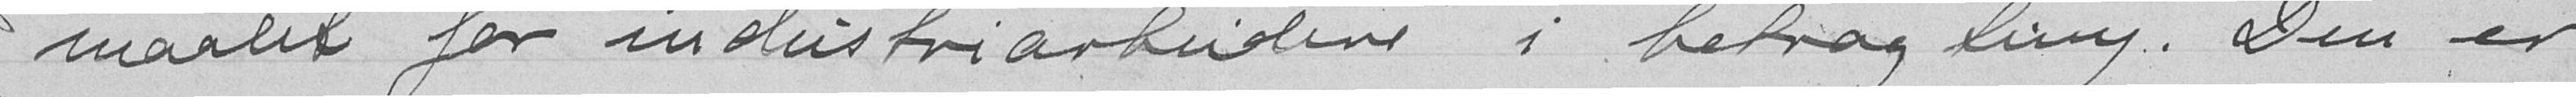maalet for industriarbeidere i betragting. Den er
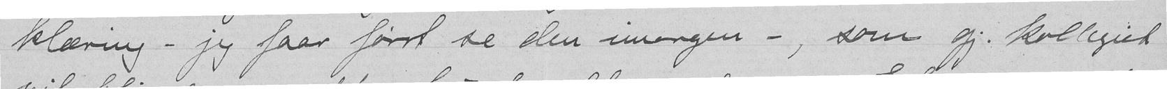klæring - jeg faar først se den imorgen -, som gj. kollegiet
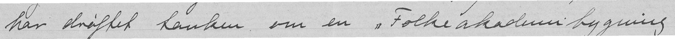har drøftet tanken om en Folkeakademibygning
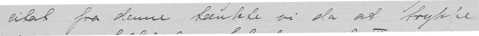citat fra denne tænkte vi dla at trykke
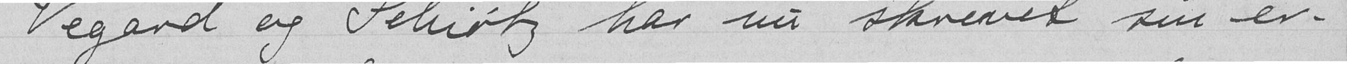Vegard og Schiøtz har nu skrevet sin er-
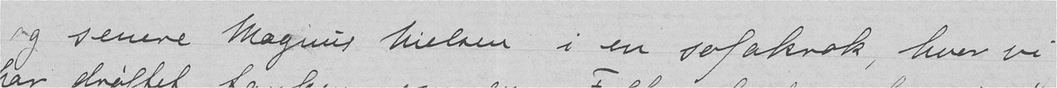og senere Magnus Nielsen i en sofakrok, hvor vi
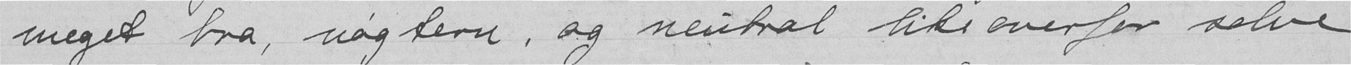meget bra, nøgtern, og neutral like overfor selve
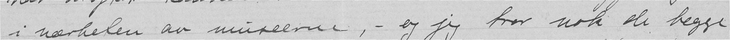i nærheten an museerne, - og jeg tror nok de begge
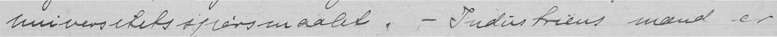Universitetsspørsmaalet. - Industriens mænd er
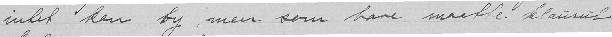intet kan by, men som bare maatte klausul
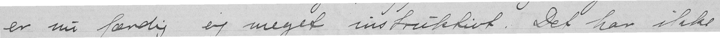er nu færdig og meget instruktivt. Det har ikke
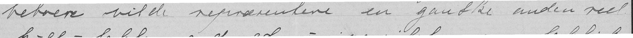beboere vilde repræsentere en ganske anden reel
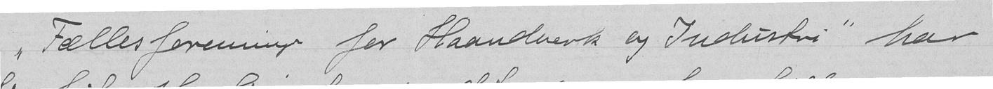„Fællesforeninge for Haandværk og Industri" har
